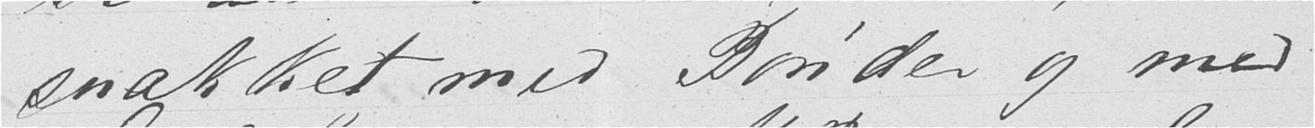snakket med Bønder og med
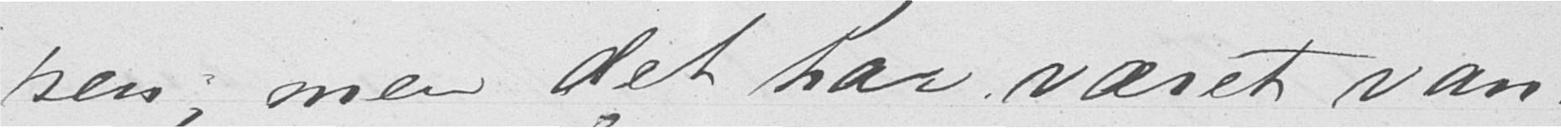ren; men det har været van-
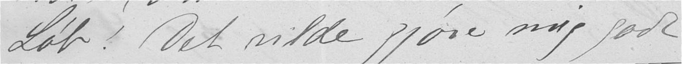Løb! Det vilde gjøre mig godt
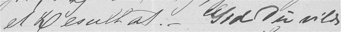et Resultat. - Gid du vilde
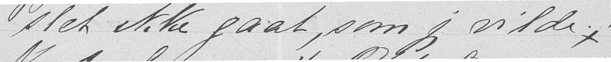slet ikke gaat, som jeg vilde;
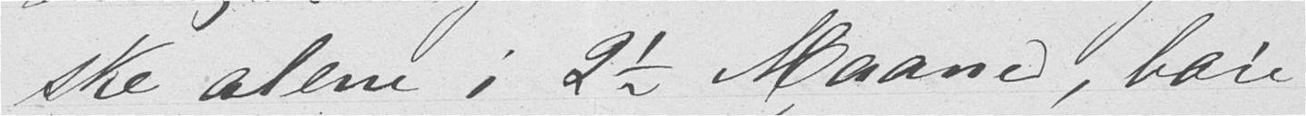ske alene i 2½ Maaned, bare
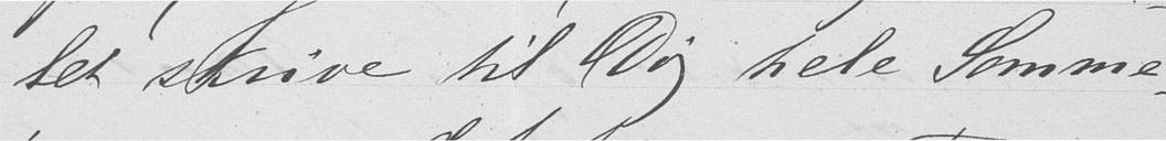let skrive til Dig hele Somme-
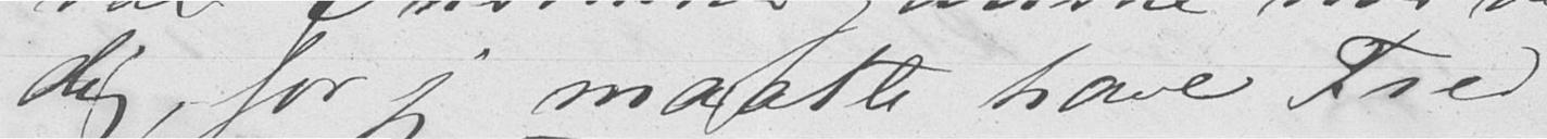dig, for jeg maatte have Fred
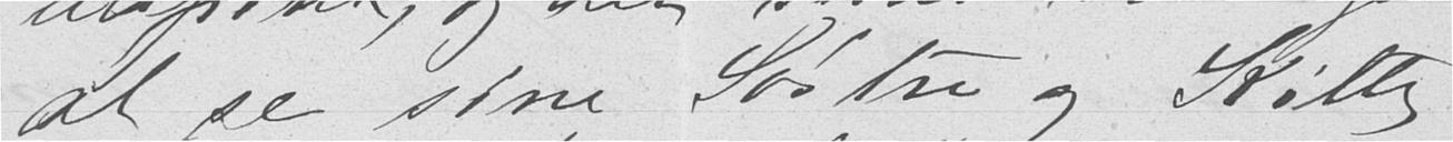at se sine Søstre og Kitty
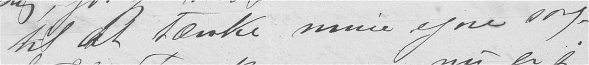til at tænke mine egne sorg-
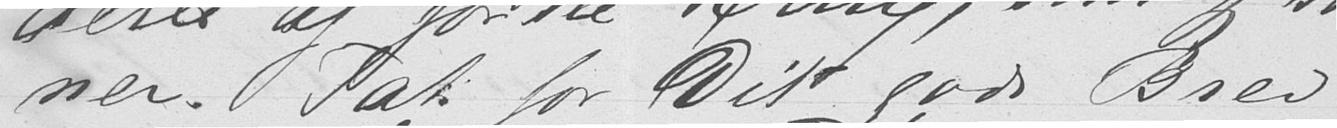ner. Tak for Dit gode Brev
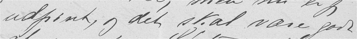udpint, og det skal være godt
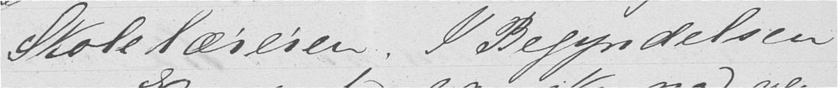Skolelæreren. I Begyndelsen
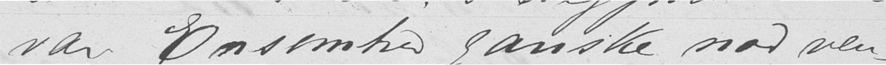var Ensomhed ganske nødven-
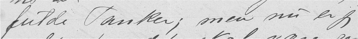fulde Tanker; men nu er jeg
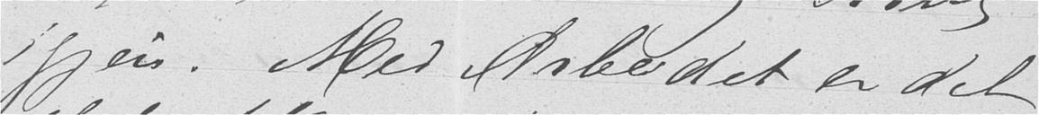igjen. Med Arbedet er det
